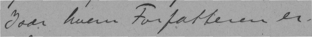Især hvem Forfatteren er.
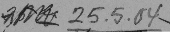25.5.04
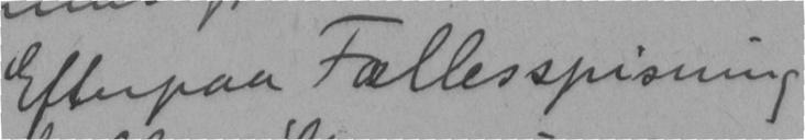Efterpaa Fællesspisning
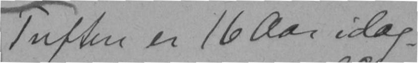Tuften er 16 Aar idag.
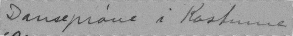Danseprøve i Kostume
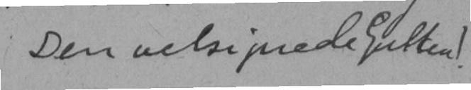Den velsignede gulten!
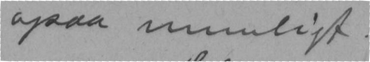ogsaa umuligt.
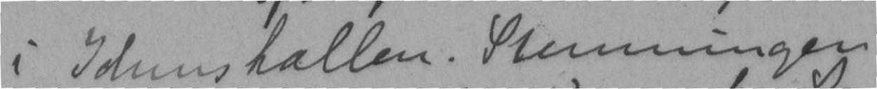i Idunshallen. Stemningen
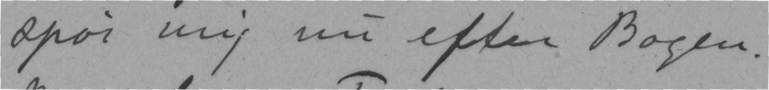spør mig nu efter Bogen.
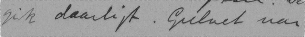gik daarligt. Gulvet var
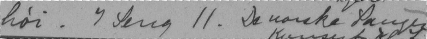høi. I Seng 11. De norske Sanger
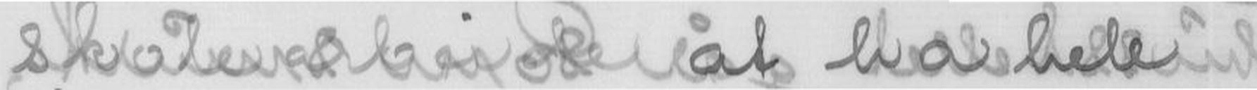skolearbeide at ha hele
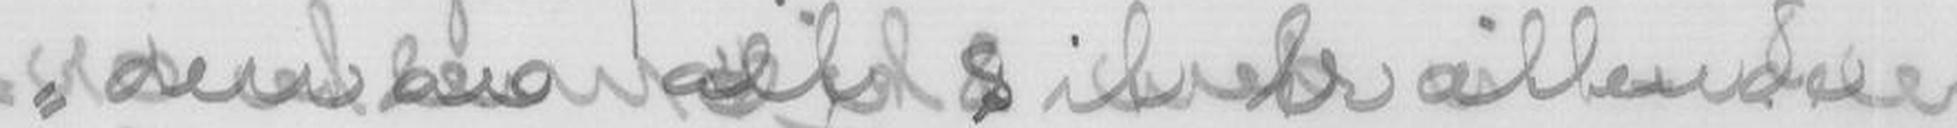den av alt sit trættende
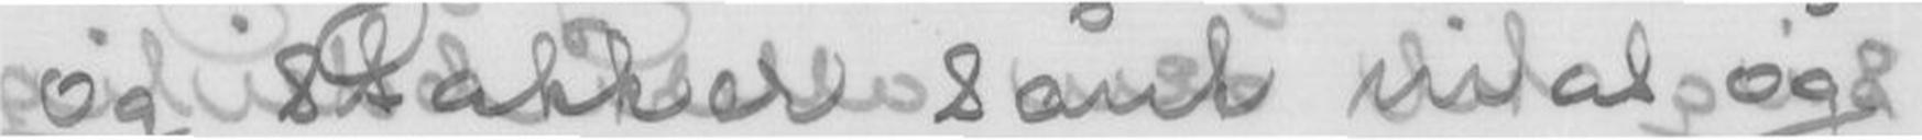og stakker sånt mas og
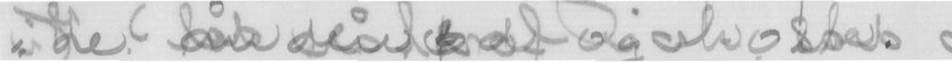de åndenød og hoste.
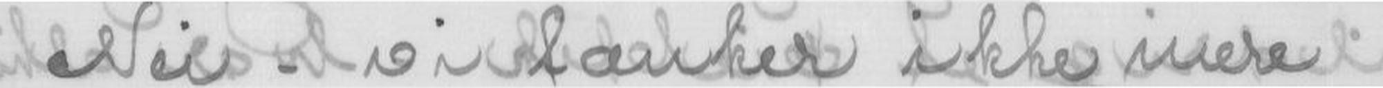Nei - vi tænker ikke mere
